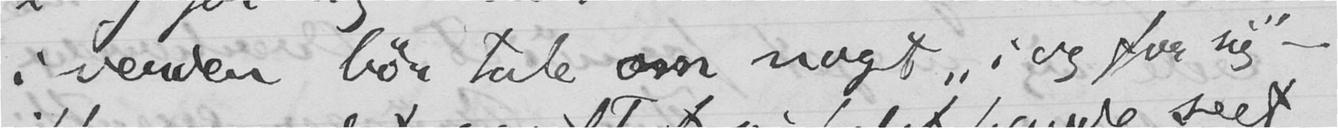i verden bør tale om noget "i og for sig" -
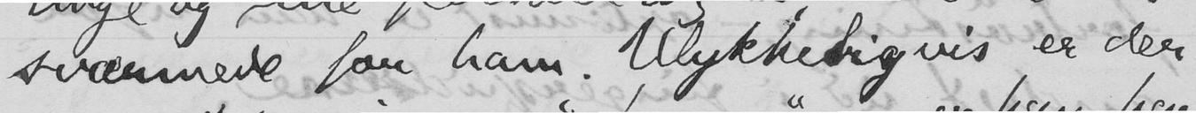sværmede for ham. Ulykkeligvis er der
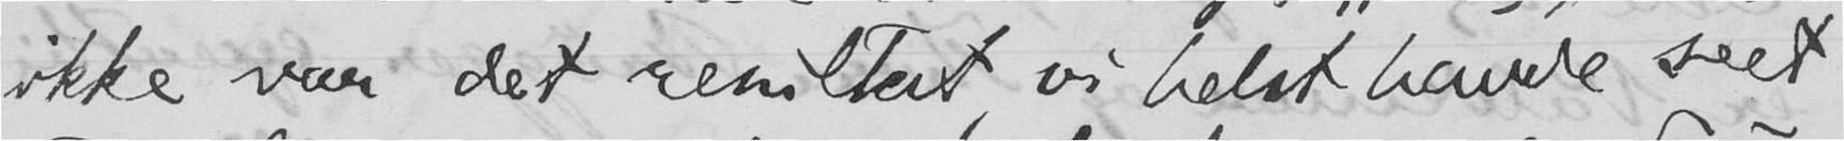ikke var det resultat, vi helst havde seet.
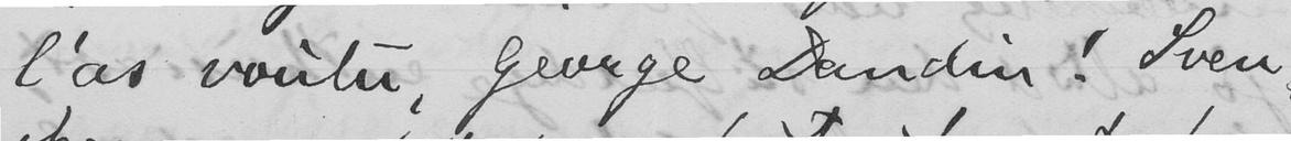l'as voulu, George Dandin! Sven-
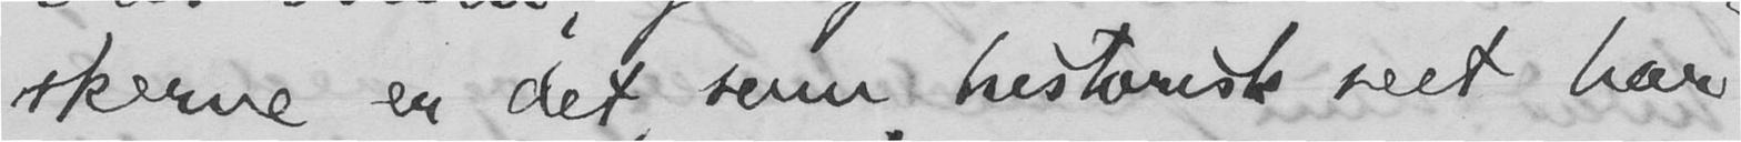skerne er det, som historisk seet har
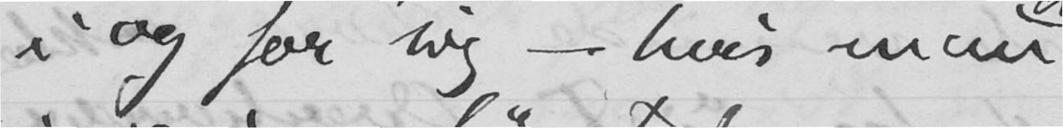i og for sig - hvis man
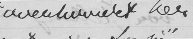overhovedet her
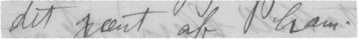det pænt af ham.
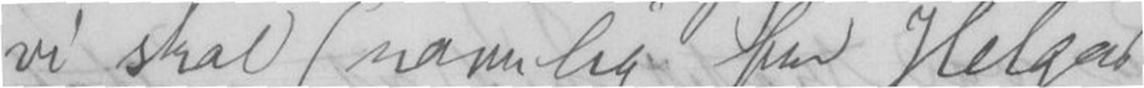vi skal (næmlig for Helgas
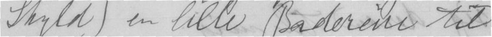Skyld) en lille Badereise til
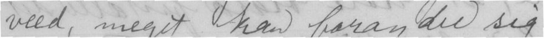veed, meget kan forandre sig
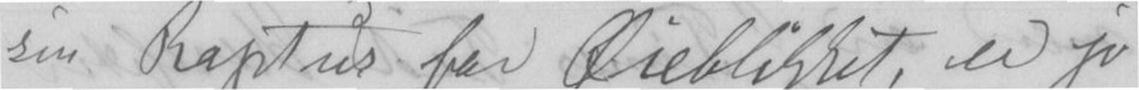sin Raptus for Øieblikket, er jo
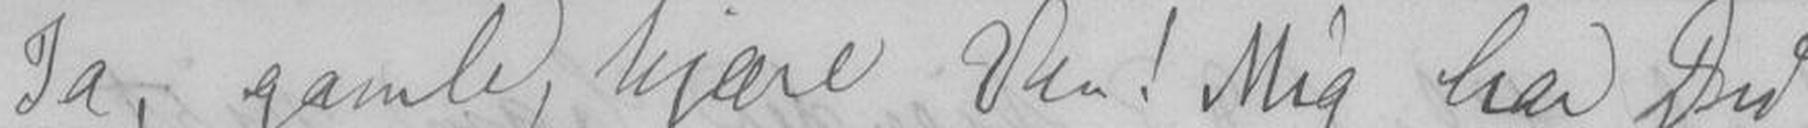Ja, gamle, kjære Ven ! Mig har Du
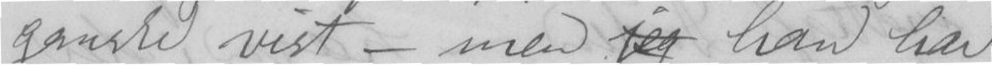ganske vist - men han har
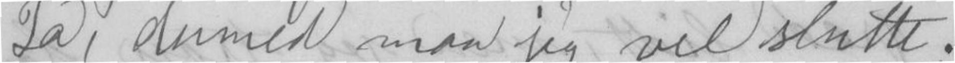Ja, dermed maa jeg vel slutte;
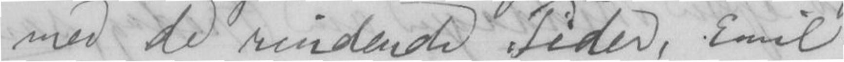med de rindende Tider, Emil
